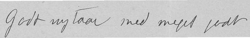Godt nytaar med meget godt
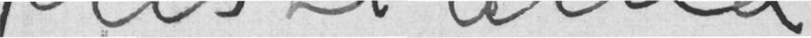Kristiania
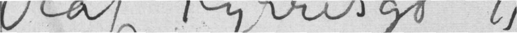Olaf Kyrres gd 11
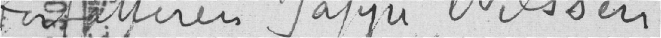Forfatteren Jappe Nilssen
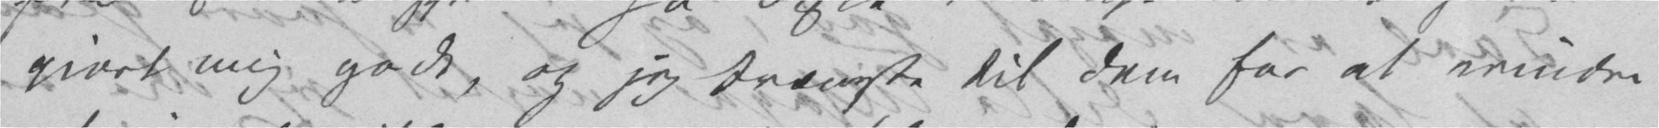gjort mig godt, og jeg trængte til dem for at erindre
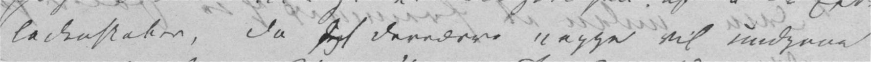ladenskaber, da det desværre neppe vil undgaae
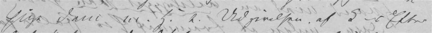sige Dem m. H. t. Udgivelsen af C-s Efter-
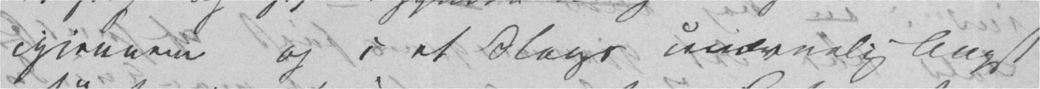igjennem og i et Slags unævnelig Angst
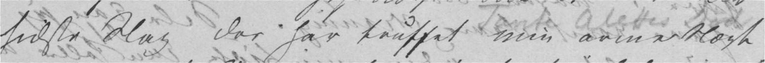sidste Slag der har truffet min arme Slægt
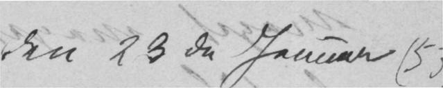Den 23de Januar
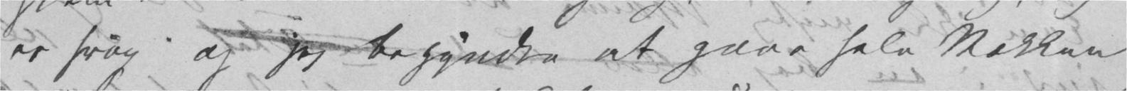er svag, og jeg begyndte at gaae hele Rækken
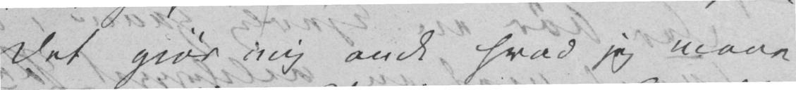Det gjør mig ondt hvad jeg maae
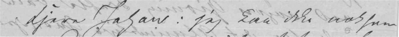Kjere Johan: jeg kan ikke noksom
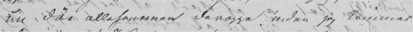nu døe allesammen deroppe inden jeg kommer
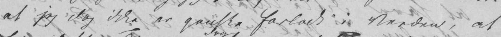at jeg dog ikke er ganske forladt i Verden, at
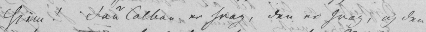hjem! Fru Colban er svag, den er svag, og den
